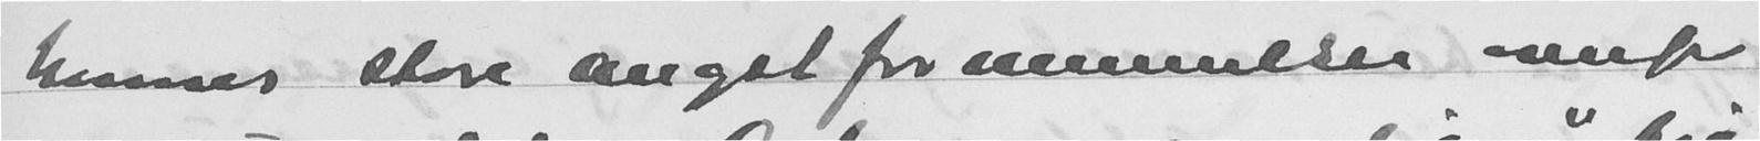Hennes store angst for mennesker ovenf
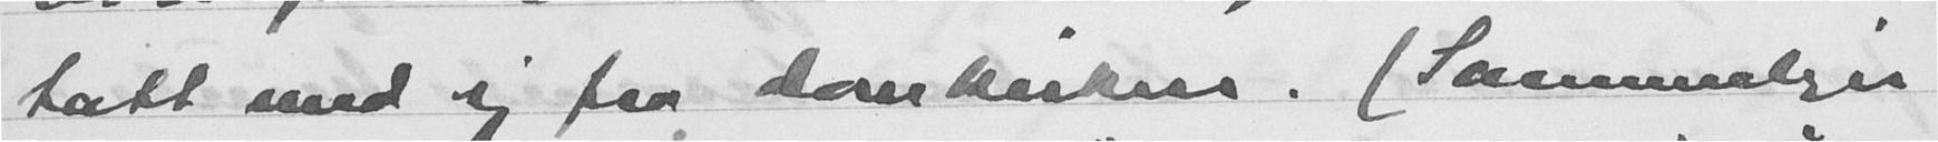tatt med sig fra Dankiskers. (Sammenlign
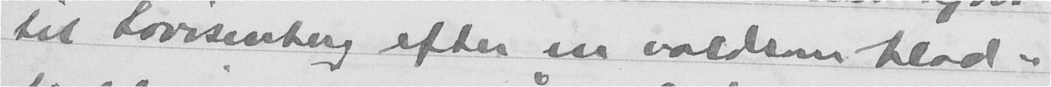til Lovisenberg efter en voldsom blod-
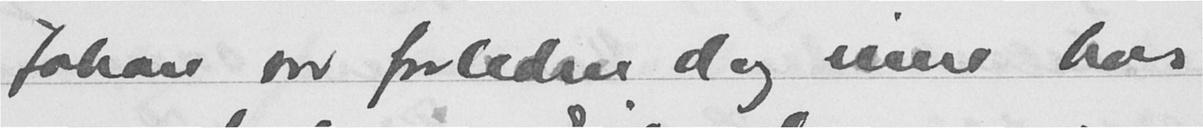Johan var forleden dag inne hos
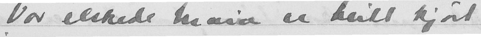Vor elskede Maia er blitt kjørt
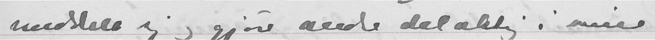meddele sig og gjøre andre delaktig i sine
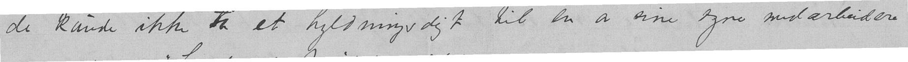de kunde ikke ta et hyldningsdigt til en av sine egne medarbeidere
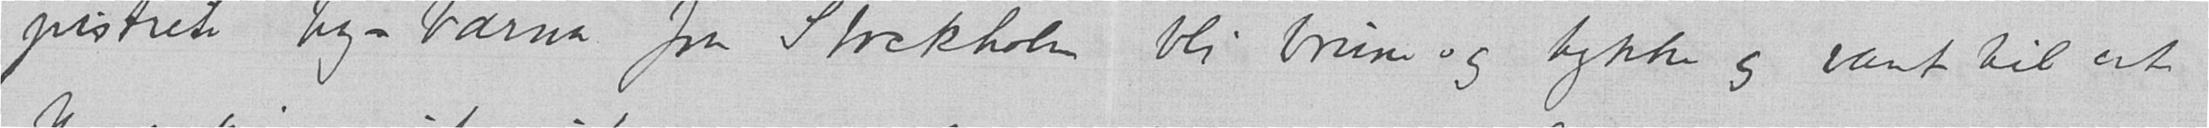pistrete by-barna fra Stockholm bli brune og tykke og vant til at
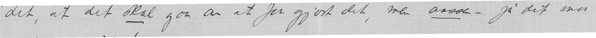det, at det skal gaa an at faa gjort det, men aassen – ja det maa
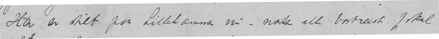Her er stilt paa Lillehammer nu – næsten alle bortreist. Jeg skal
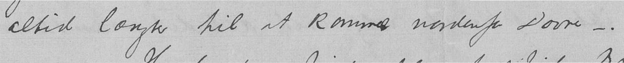altid længter til at komme nordenfor Dovre –.
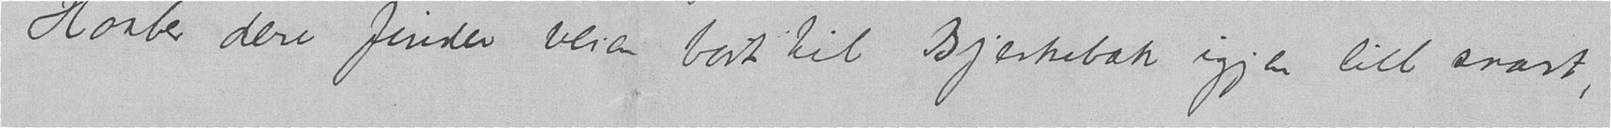Haaber dere finder veien bort til Bjerkebæk igjen litt snart,
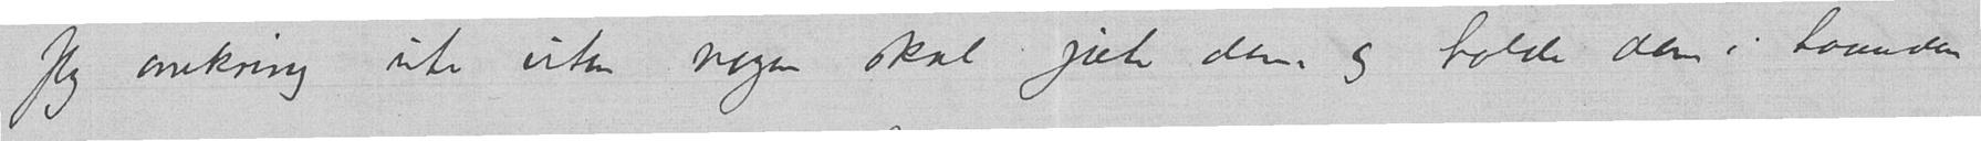fly omkring ute uten nogen skal gjete dem og holde dem i haanden
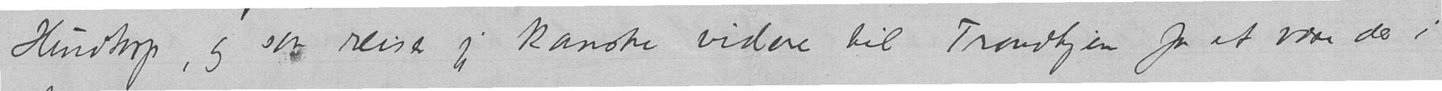Hundtorp, og saa reiser jeg kanske videre til Trondhjem for at være der i
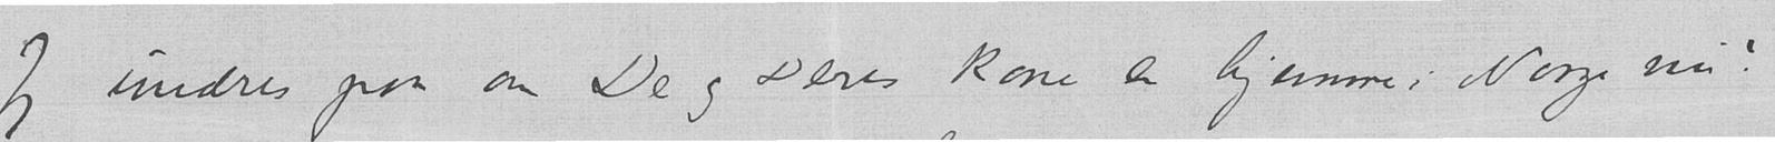Jeg undres paa om De og Deres kone en hjemme i Norge nu?
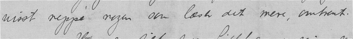visst neppe nogen som læser det mere, omtrent.
